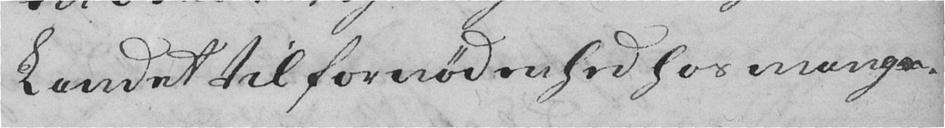Landet til fornødenhed hos mange.
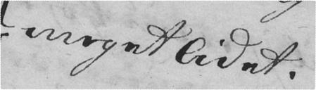meget lidet.
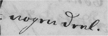nogen deel.
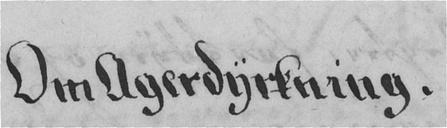Om Agerdyrkning.
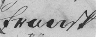Fransk
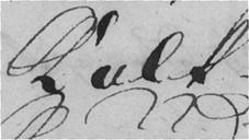Kalk
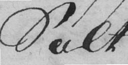Salt
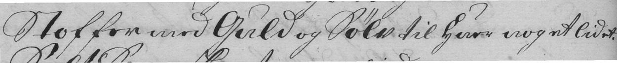Stoffer med Guld og Sølv til Huer noget lidet
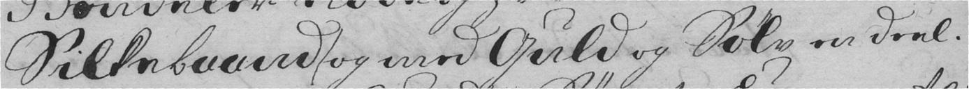Silkebaand og med Guld og Sølv en deel.
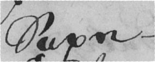Sage
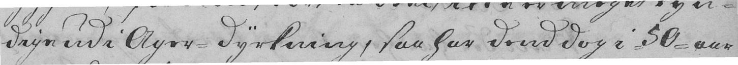dige ud i Ager-dyrkning, saa har dend dog i 50 aar
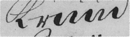Kruud
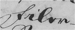Filer
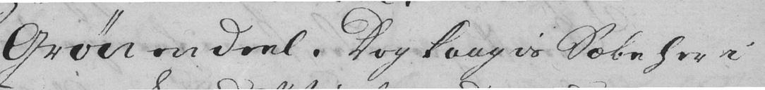Grøn en deel. Dog kaagis Sæbe her i
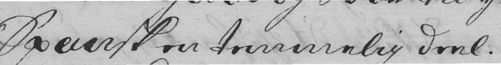Spansk en temmelig deel
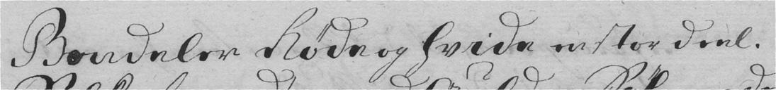Bendeler Røde og hvide en stor deel.
